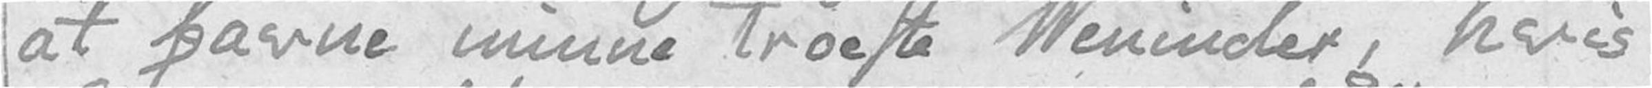at favne minne troeste Veninder, hvis
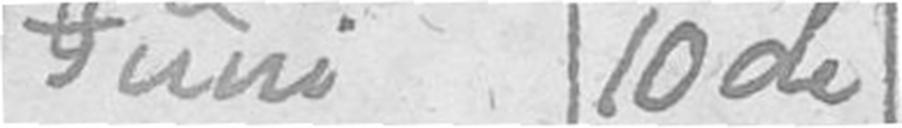Juni 10de
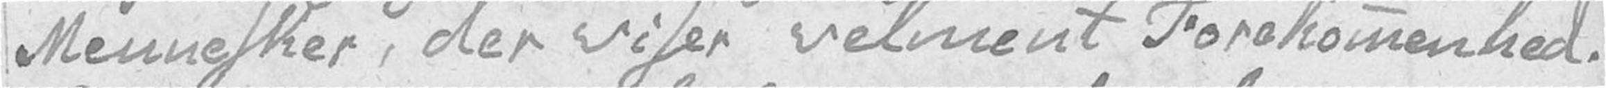Mennesker, der viser velment Forekommenhed.
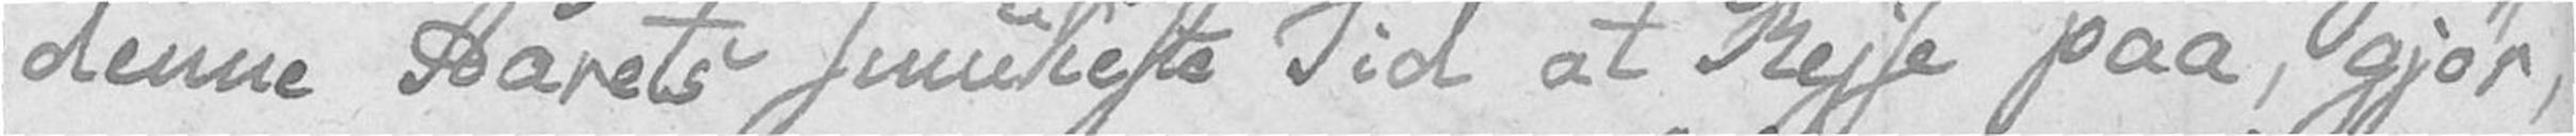denne Aarets smukeste Tid at Rejse paa, gjør,
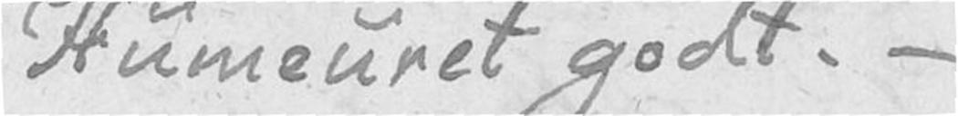Humeuret godt. –
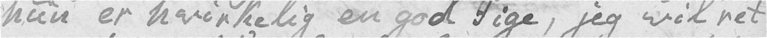hun er hvirkelig en god Pige, jeg vil ret
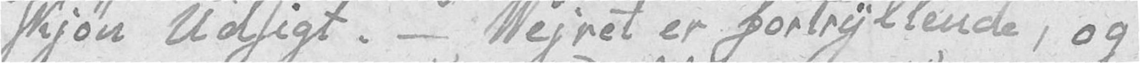skjøn Udsigt. – Vejret er fortryllende, og
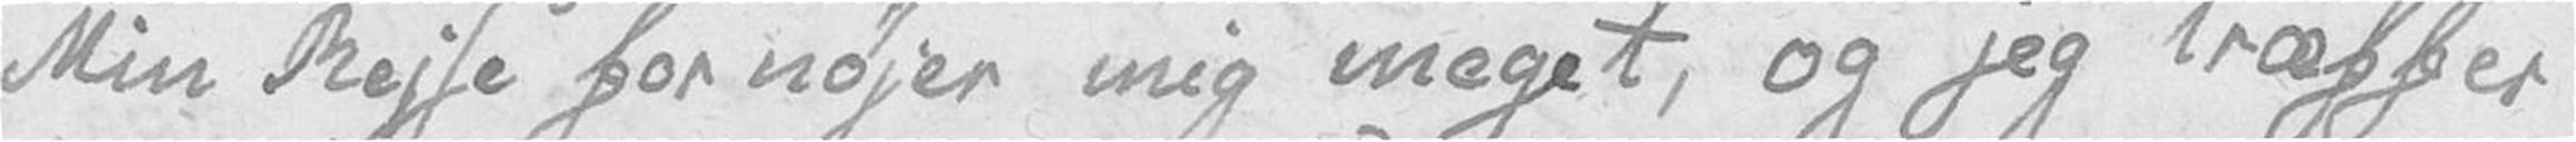Min Rejse fornøjer mig meget, og jeg træffer
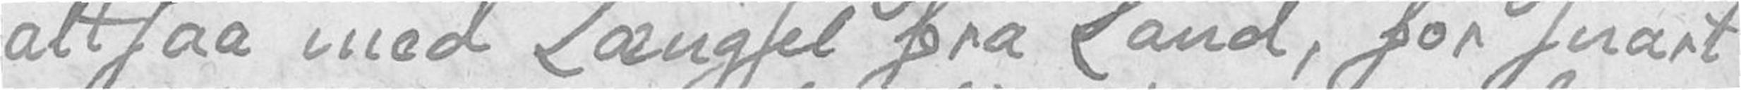altsaa med Længsel fra Land, for snart
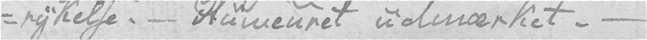-rykelse. – Humeuret udmærket. –
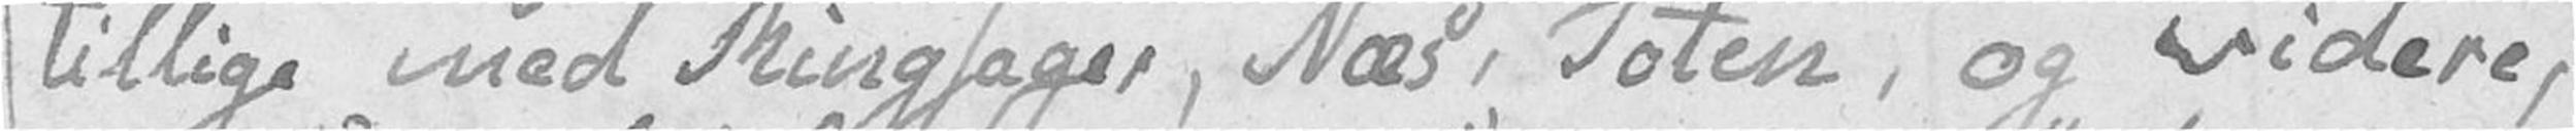tillige med Ringsager, Næs, Toten, og videre,
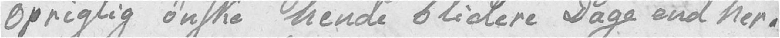Oprigtig ønske hende blidere Dage end her.
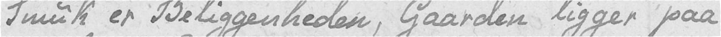Smuk er Beliggenheden, Gaarden ligger paa
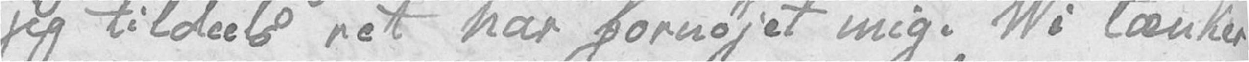jeg tildeels ret har fornøjet mig. Vi tænker
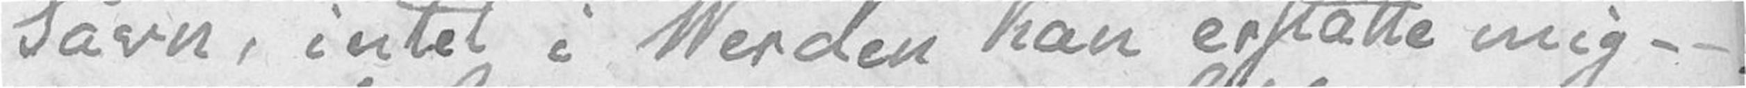Savn, intet i Verden kan erstatte mig. –
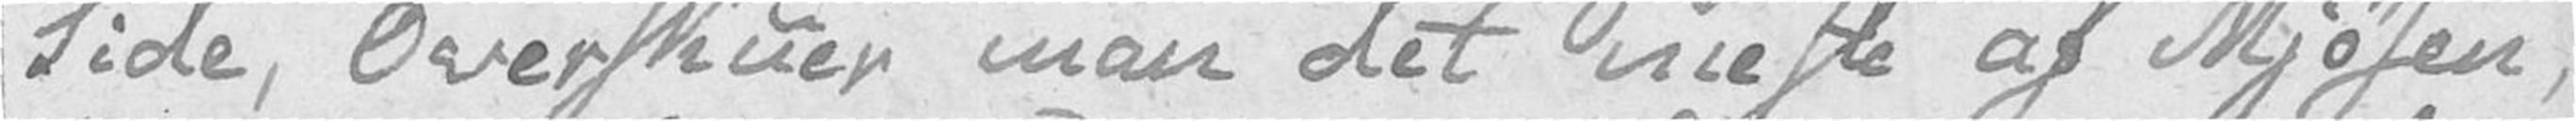Side, Overskuer man det meste af Mjøsen,
